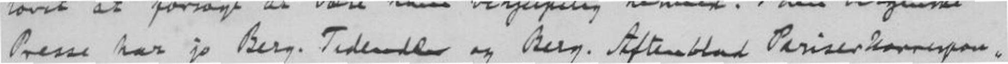Presse har jo Berg. Tidende og Berg. Aftenblad Pariserkorrespon-
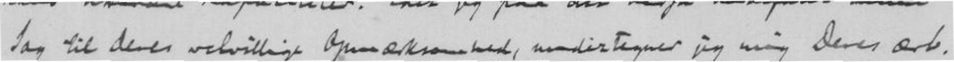Sag til Deres velvillige Opmærksomhed, undertegner jeg mig Deres Ærb.
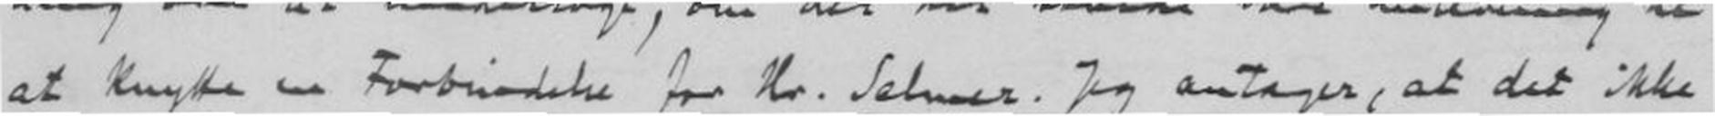at Knytte en Forbindelse for Hr. Selmer. Jeg antager, at det ikke
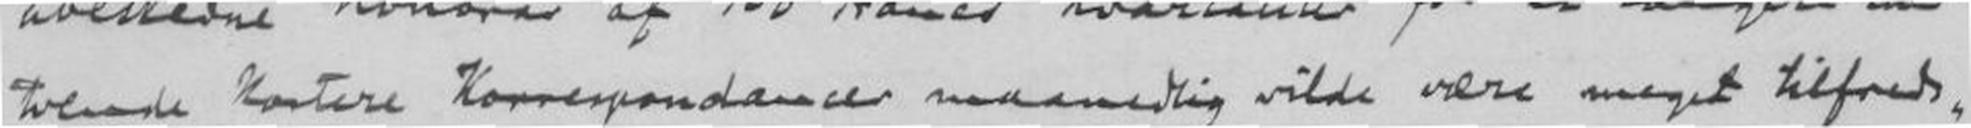tvende Kortere Korrespondancer maanedlig vilde være meget tilfreds-
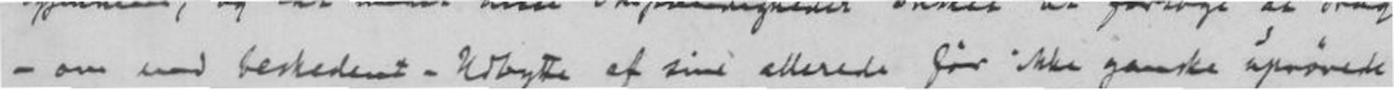- om med beskedent - Udbytte af sine allerede før ikke ganske uprøvede
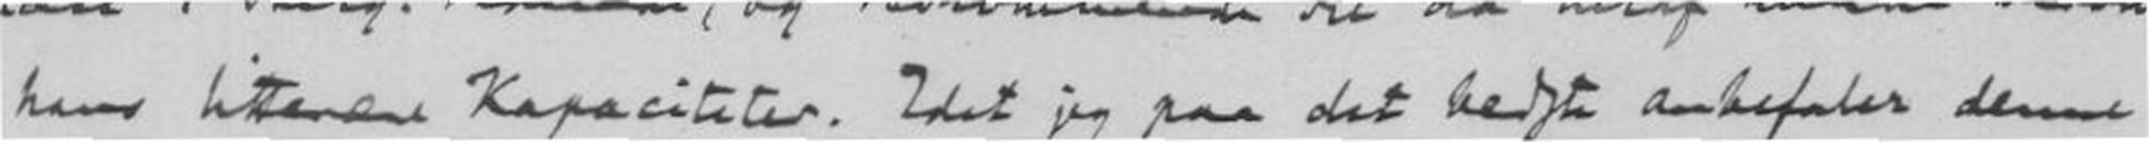hans litterære Kapaciteter. Idet jeg paa det bedste anbefaler denne
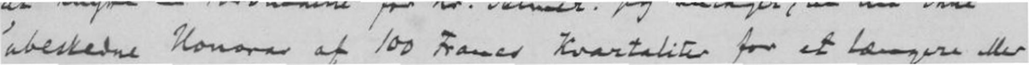ubeskedne Honorar af 100 Francs Kvartaliter for et længere eller
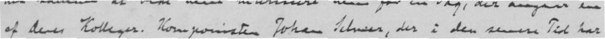af Deres Kolleger. Komponisten Johan Selmer, der i den senere Tid har
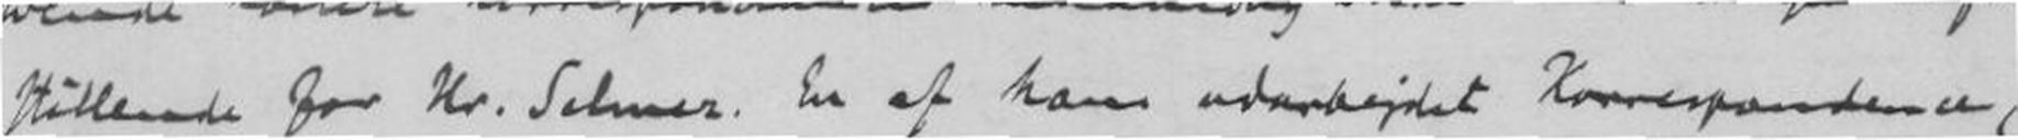stillende for Hr. Selmer. En af hans udarbejdet Korrespondancer,
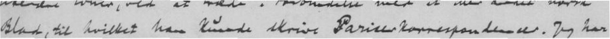Blad, til hvilket han kunde skrive Pariserkorrespondenser. Jeg har
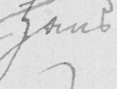Hans
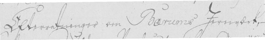Efterretninger om Bærums Jernværk, -
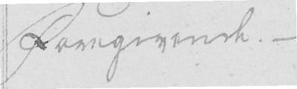Foregivende. -
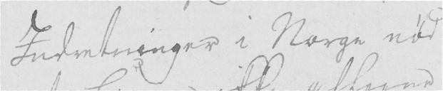Indretninger i Norge nød
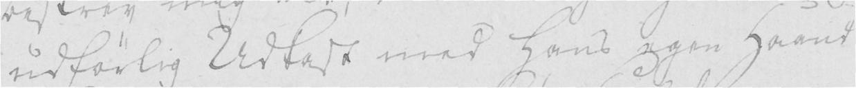udførlig Udkast med hans egen Haand
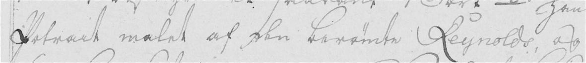Portrait malet af den berømte Reynolds, og
